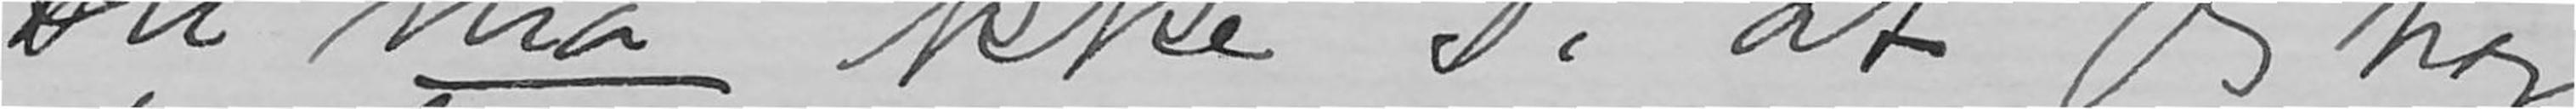Du må ikke si at jeg har
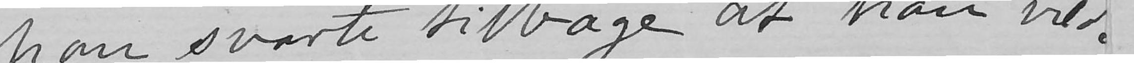han svarte tilbage at han vilde
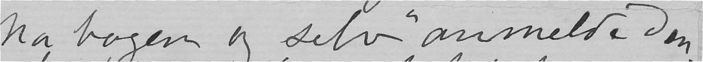ha bogen og "selv" anmelde den.
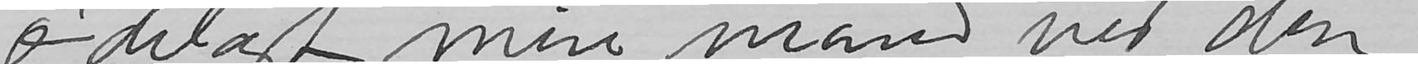ødelagt min mand ved den
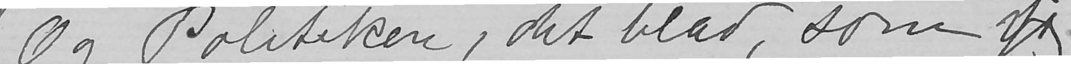Og Politiken, det blad, som jeg dog
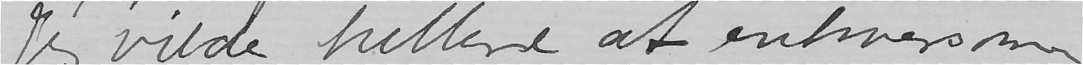Jeg vilde hellere at enhversom-
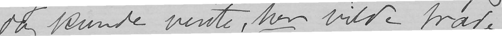kunde vente, her vilde træde
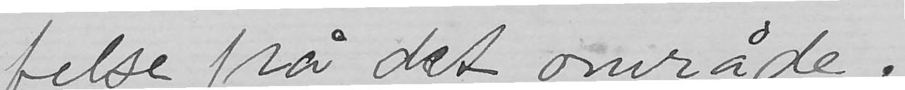felse på det område.
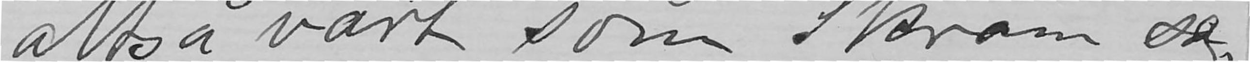altså vært som Skram sa,
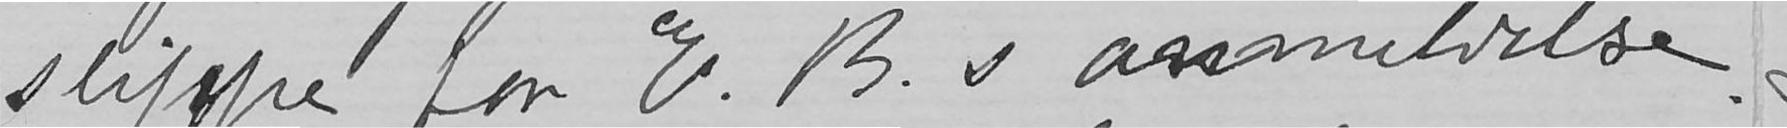slippe for E.B.s anmeldelse.
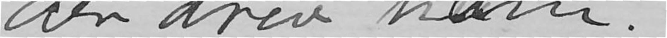der drev ham.
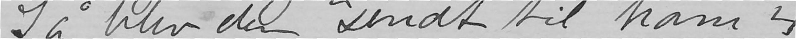Så blev den sendt til ham 4
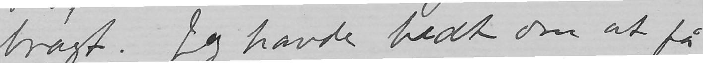bragt. Jeg havde bedt om at få
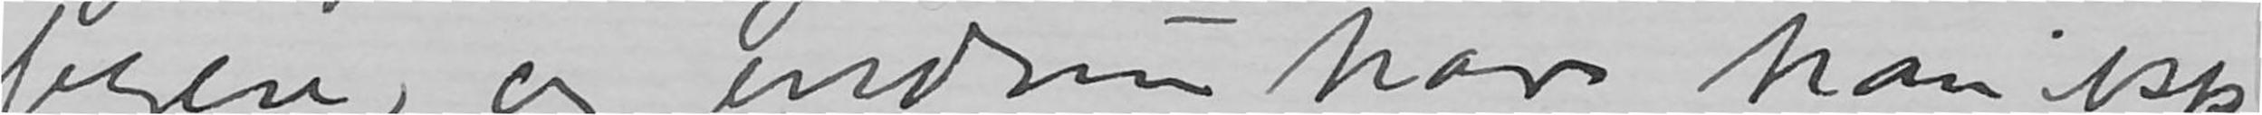byen, og endnu har han ikke
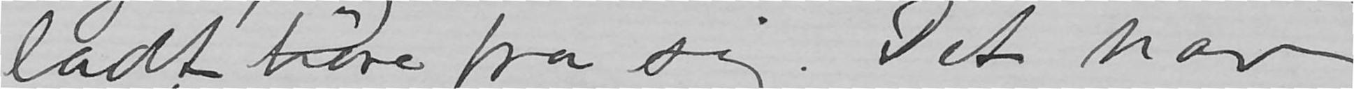ladt høre fra sig. Det har
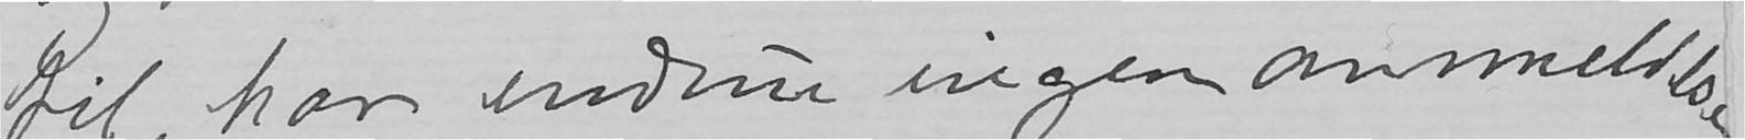til, har endnu ingen anmeldelse
